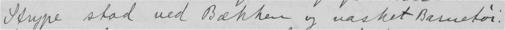Strype stod ved Bækken og vasket Barnetøi.
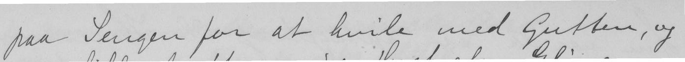paa Sengen for at hvile med Gutten, og
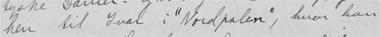hen til Ivar i "Nordpolen", hvor han
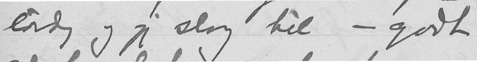lørdag og jeg slog til - godt
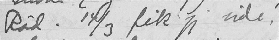Rød. 14/3 fik jeg vide,
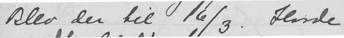Blev der til 16/3. Havde
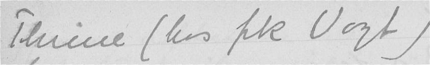Thrine (hos frk Vogt)
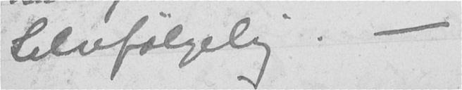Selvfølgelig. -
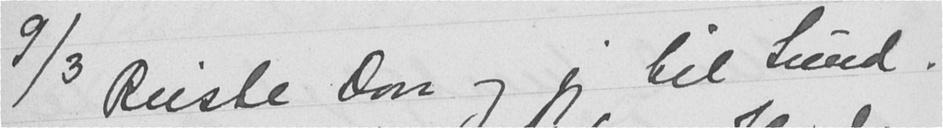9/3 Reiste Don og jeg til Sund.
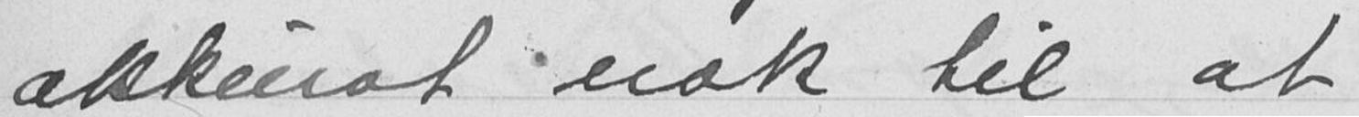akkurat nok til at
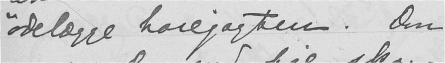ødelægge harejagten. Don
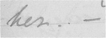her. -
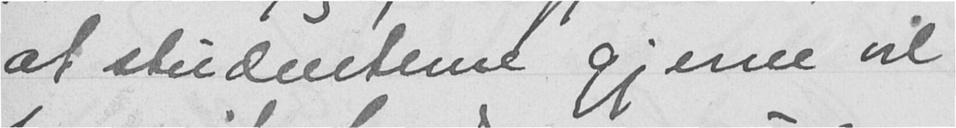at studenterne gjerne vil
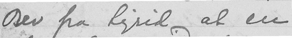Brev fra Sigrid at en
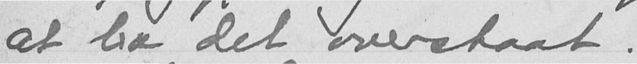at ha det overstaat.
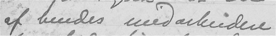af hendes medarbeidere
